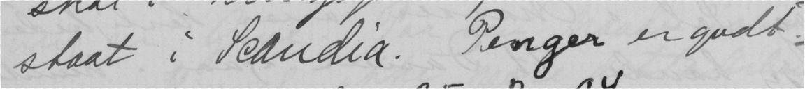staat i Scandia. Penger er godt.
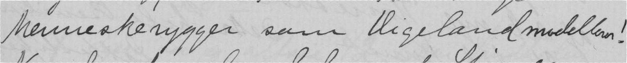Menneskerygger som Vigeland modellerer!
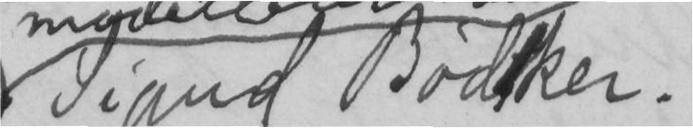Sigurd Bødker.
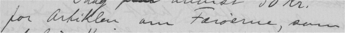for Artiklen om Færøerne, som
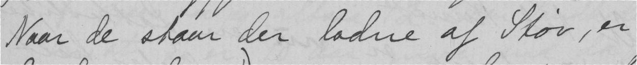Naar de staar der lodne af Støv, er
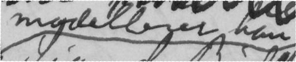modellerer han
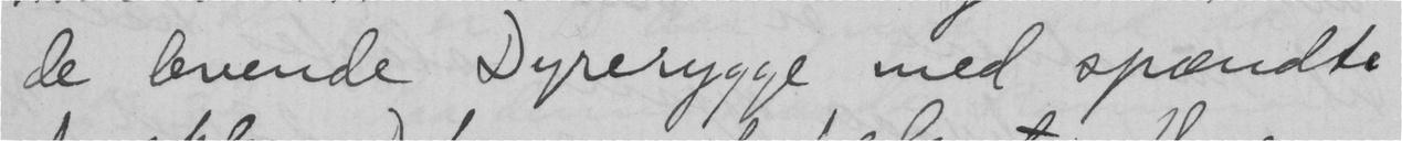de levende Dyrerygge med spændte
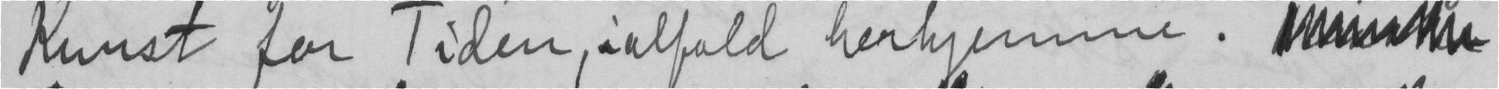Kunst for Tiden, ialfald herhjemme.
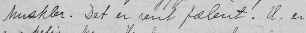Muskler. Det er rent fælent. V. er
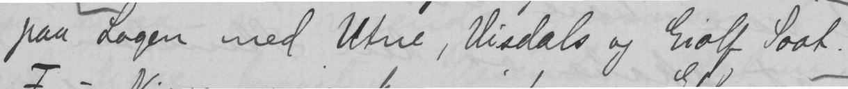paa Logen med Utne, Visdals og Eiolf Soot.
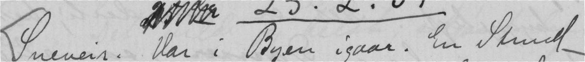Sneveir. Ver i Byen igaar. En Stund
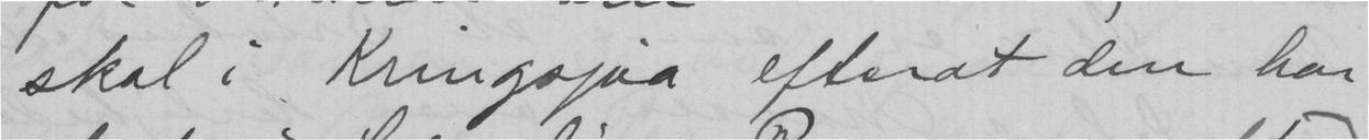skal i Kringsjaa efterat den har
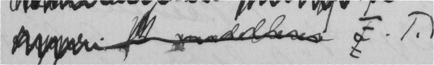T.
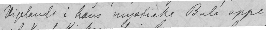Vigelands i hans mystiske Bule oppe
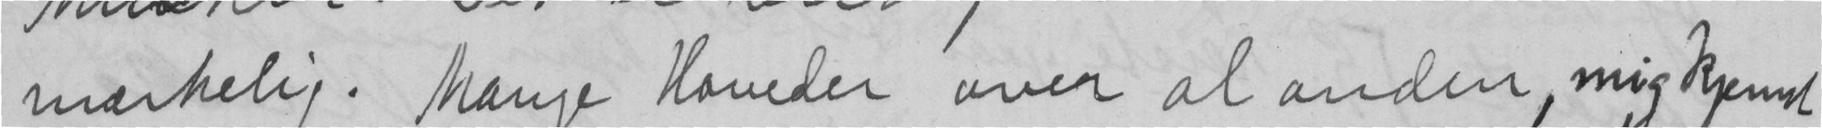mærkelig. Mange Hoveder over al anden, mig kjennt
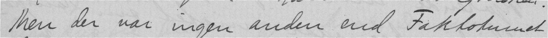Men der var ingen anden end Faktotumet
